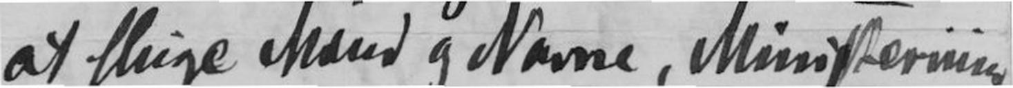at sluge Mænd og Navne, Ministerium
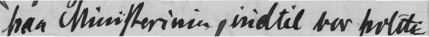paa Ministerium indtil vor polit-
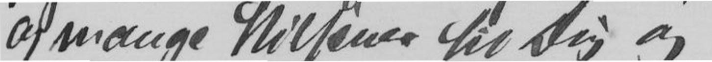og mange Hilsener til Dig og
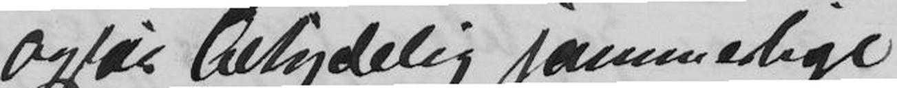ogsaa Betydelig jammerlige
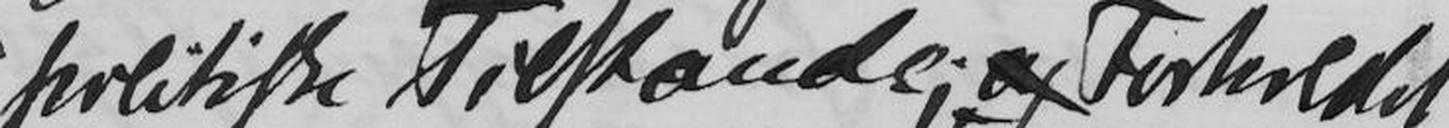politiske Tilstande; og Forholdet
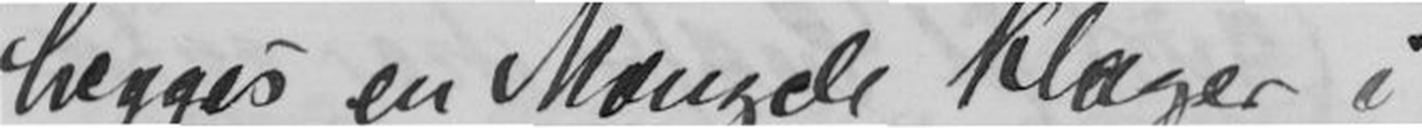lægges en Mængde klager i
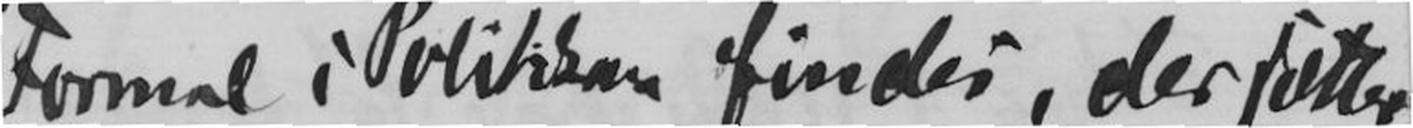Formal i Politiken findes, der sitter
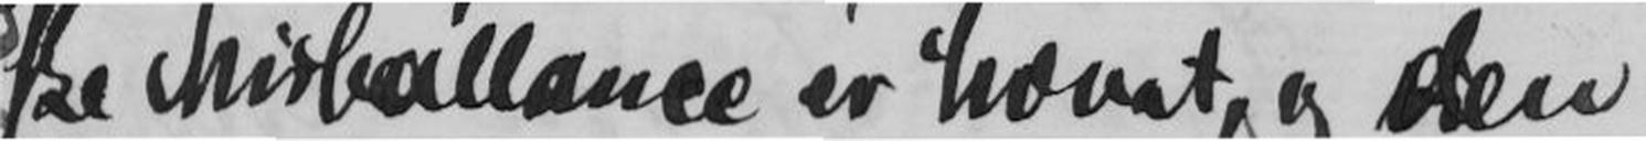tiske Misballance er hævet, og den
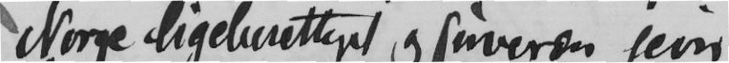Norge ligeberettiget og suveræn jevn
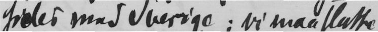sides med Sverige; vi maa slutte
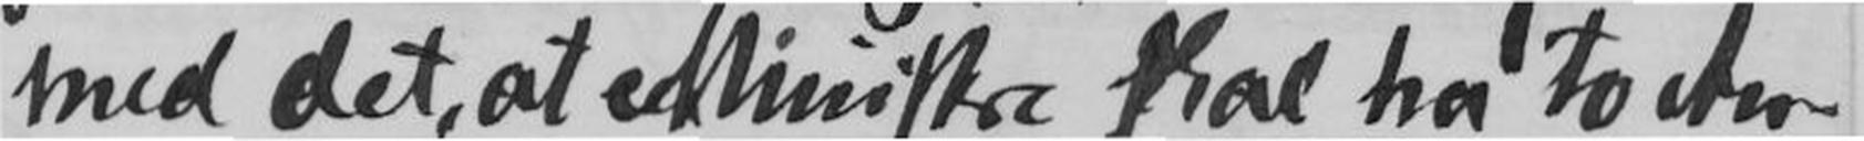med det, at Ministre skal ha to An-
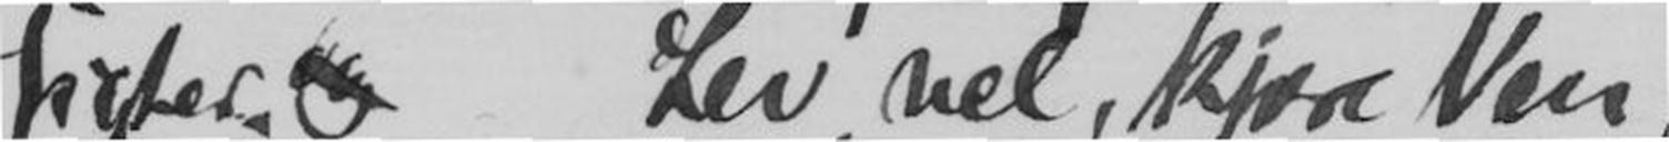sigter. - Lev vel, kjære Ven,
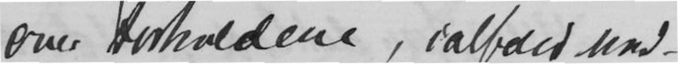over Forholdene, ialfald ned-
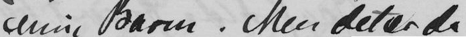mine Barn. Men det er da
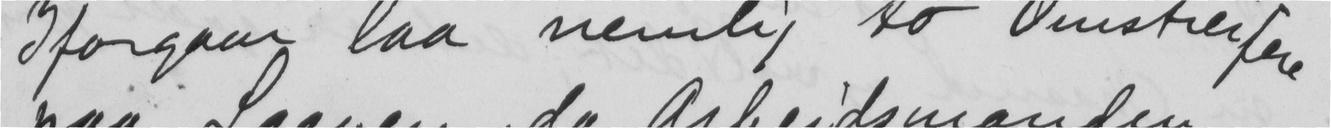I forgaar laa nemlig to Omstreifere
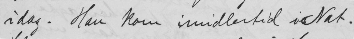idag. Han kom imidlertid i Nat.
